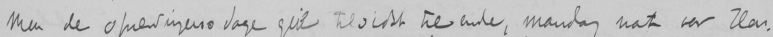Men de spændingens dage gik tilsidst til ende, mandag nat var Har-
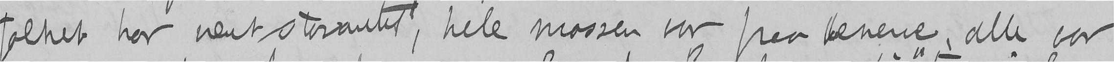folket har vært storartet, hele massen var paa benene, alle var
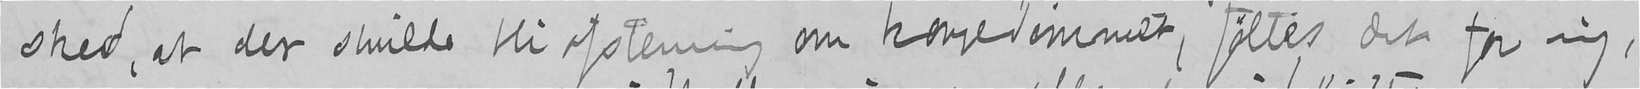sked, at der skulde bli afstemning om kongedømmet, føltes det for mig,
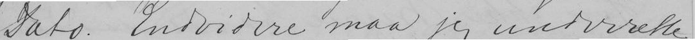Dato. Endvidere maa jeg underrette
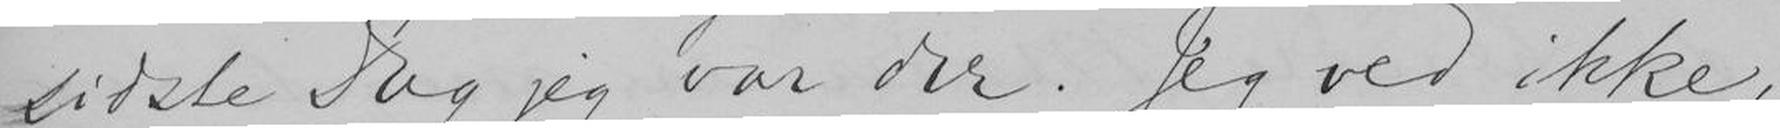sidste Dag jeg var der. Jeg ved ikke,
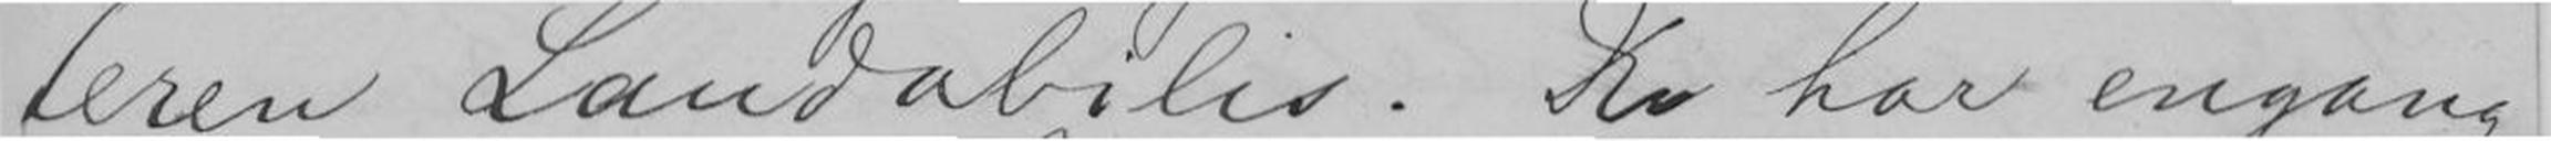teren Laudabilis. Du har engang
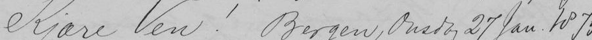Kjære Ven! Bergen, Onsdag 27 Jan. 1875.
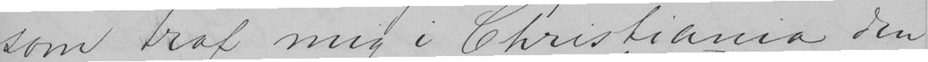som traf mig i Christiania den
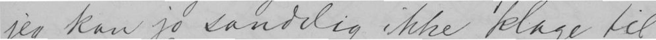jeg kan jo sandelig ikke klage til
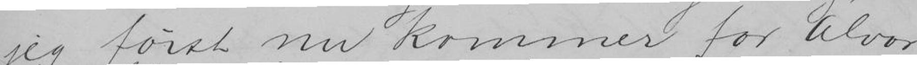jeg først nu kommer for Alvor
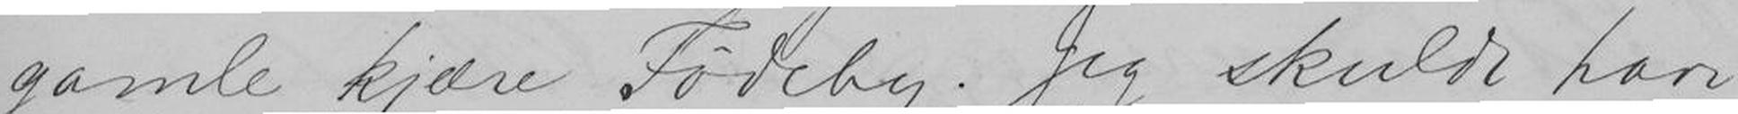gamle kjære Fødeby. Jeg skulde have
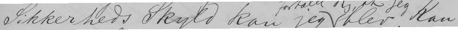Sikkerheds Skyld kan jeg blev Kan-
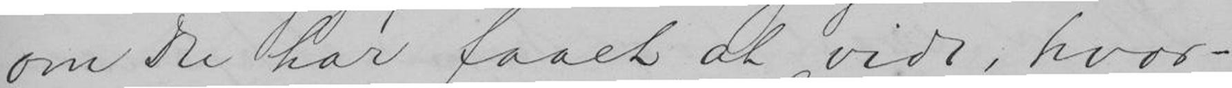om Du har faaet at vide, hvor-
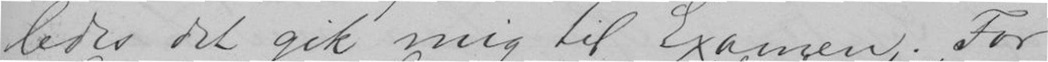ledes det gik mig til Examen. For
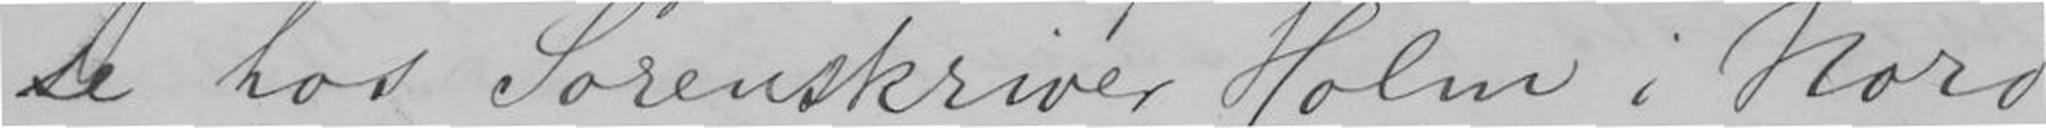se hos Sorenskriver Holm i Nord-
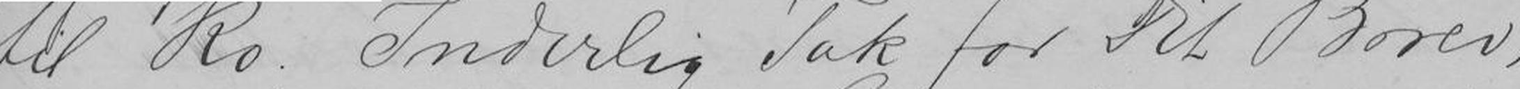til Ro. Inderlig Tak for Dit Brev,
